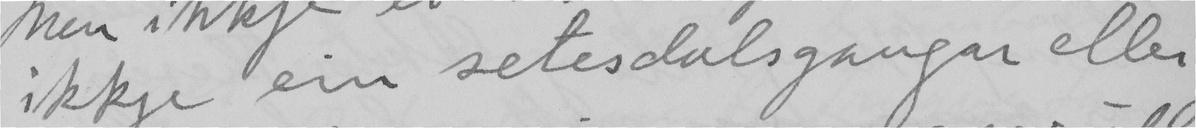ikkje ein setesdalsgangar eller
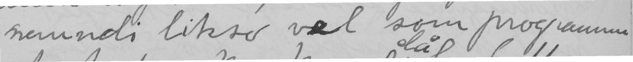nemdi likso væl som programmane
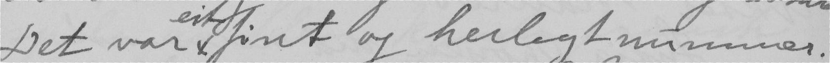Det var et fint og herlegt nummer.
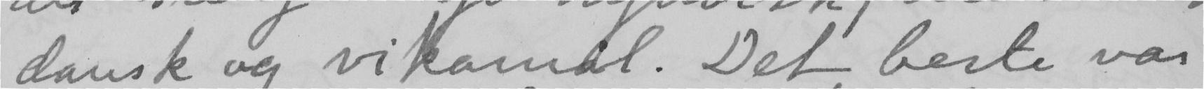dansk og vikamål. Det beste var
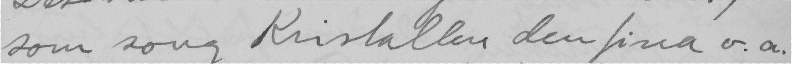som song Kristallen den fina o.a.
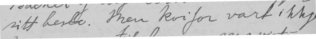sitt beste. Men kvifor vart ikkje
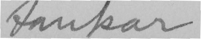tankar
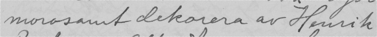morosamt dekorera av Henrik
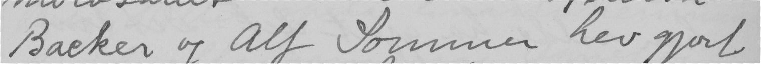Backer og Alf Sommer hev gjort
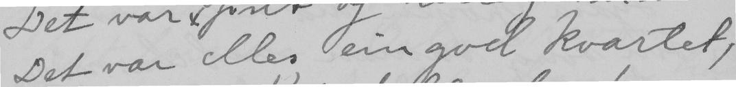Det var elles ein god kvartet,
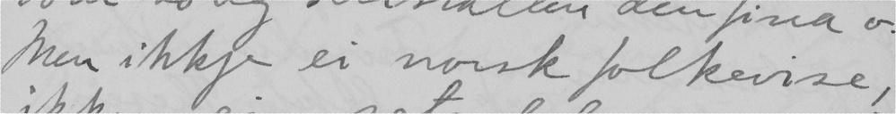Men ikkje ei norsk folkevise,
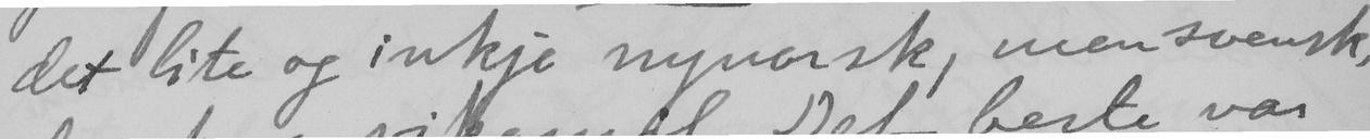det lite og inkje nynorsk, men svensk,
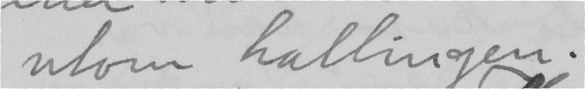utom hallingen.
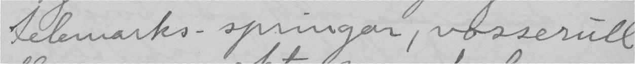Telemarks-springar, vosserull
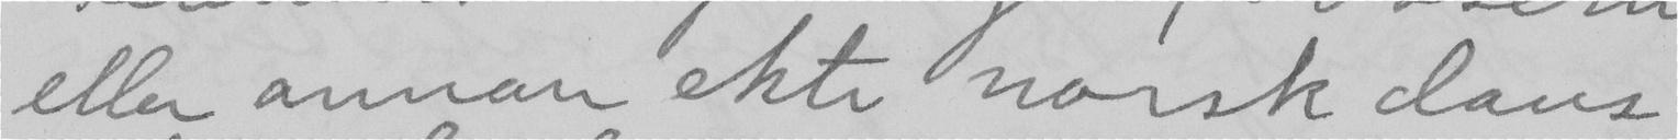eller annan ekte norsk dans
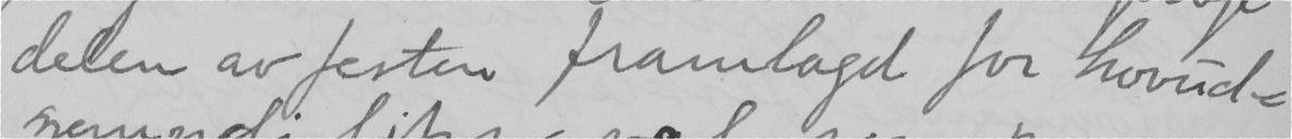delen av festen framlagd for hovud-
